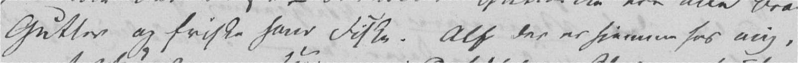Gutter og friske som Fiske. Alf der er hjemme hos mig,
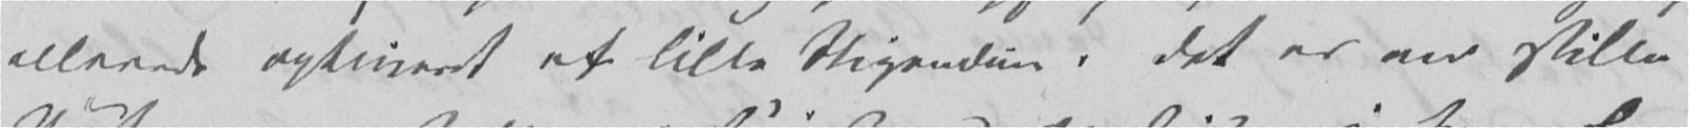allerede optineret et lille Stipendium. Det er en stille
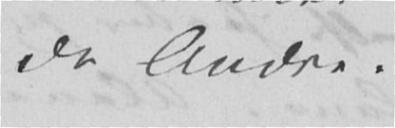de Andre.
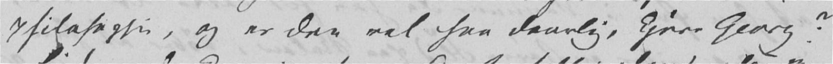philosophie, og er den vel saa daarlig, kjere Georg?
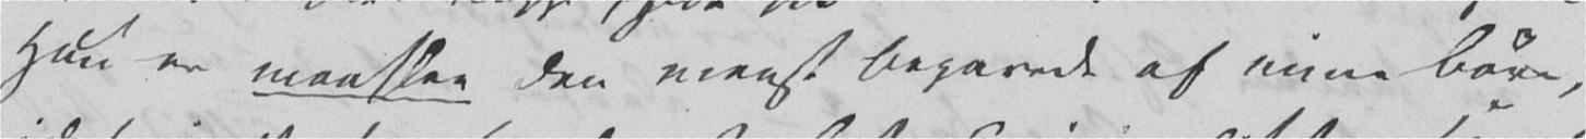Han er maaskee den meest begavede af mine Børn,
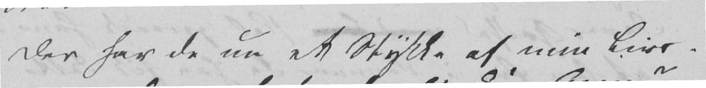Der har De nu et Stykke af min Livs-
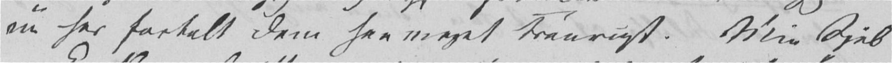nu har fortalt Dem saa meget traurigt. Min Sjel
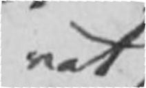ret
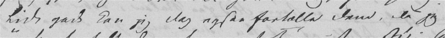Lidt godt kan jeg dog ogsaa fortælle Dem, da jeg
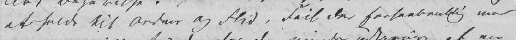at holde til Orden og Flid, Feil der forhaabentlig mere
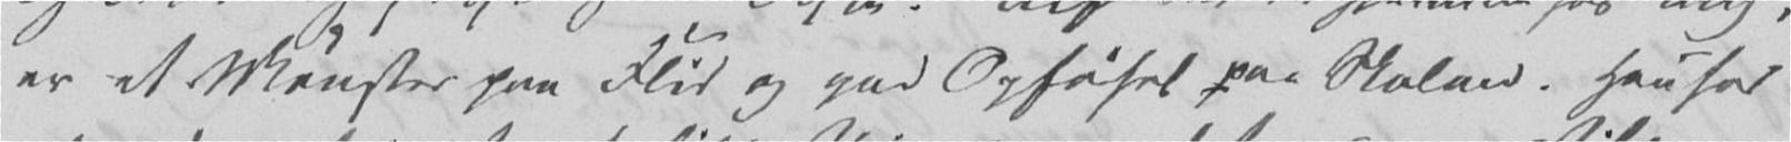er et Mønster paa Flid og god Opførsel paa Skolen. Han har
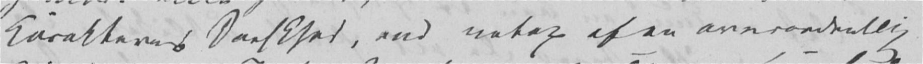Carakterens Dorskhed, end netop af en overordentlig
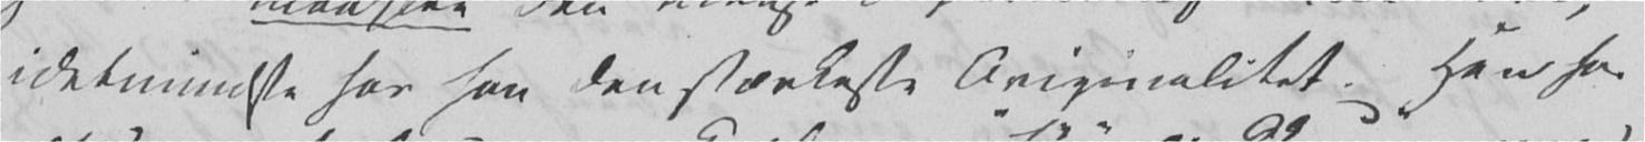idetmindste har han den stærkeste Originalitet. Han har
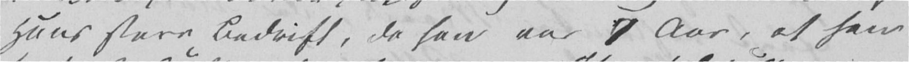Hans store Bedrift, da han var 7 Aar, at han
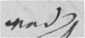ved
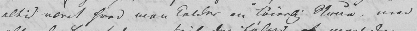altid været hvad man kalder en «løierlig Skrue», med
